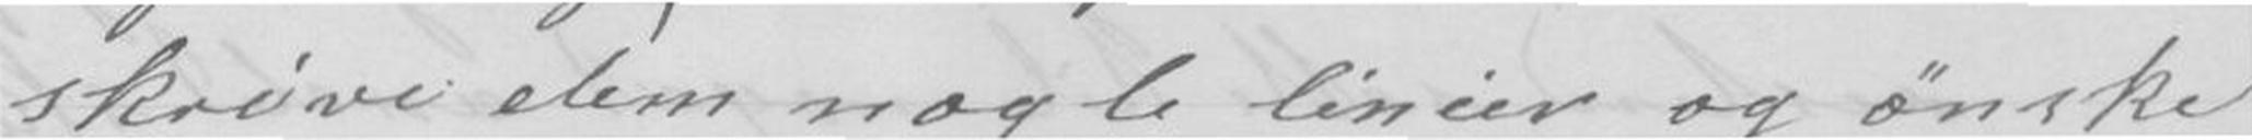skrive dem nogle linier og ønske
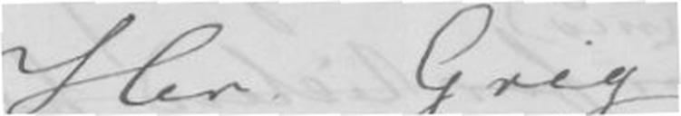Her Grig
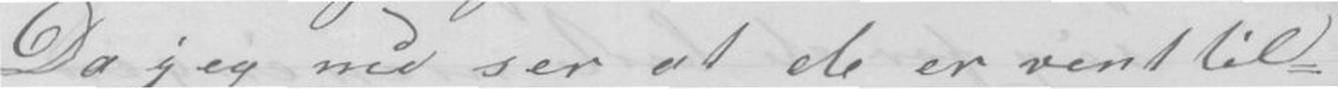Da jeg nu ser at de er vent til-
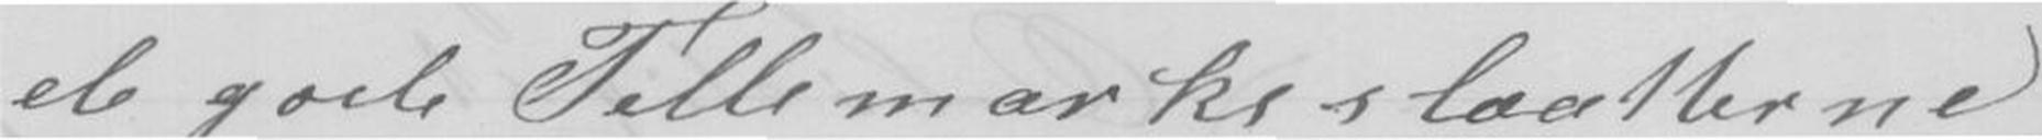de gode Tellemarksslaatterne
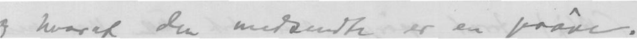og hvoraf den medsendte er en prøve.
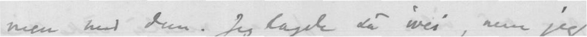men med Dem. Jeg lagede så ivei, men jeg
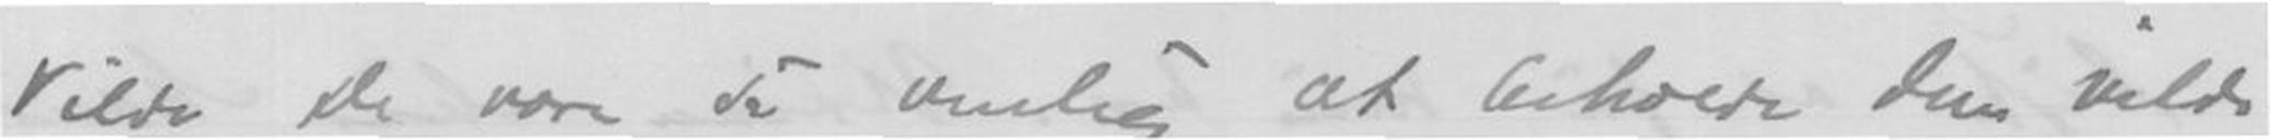Vilde De være så venlig at beholde dem vilde
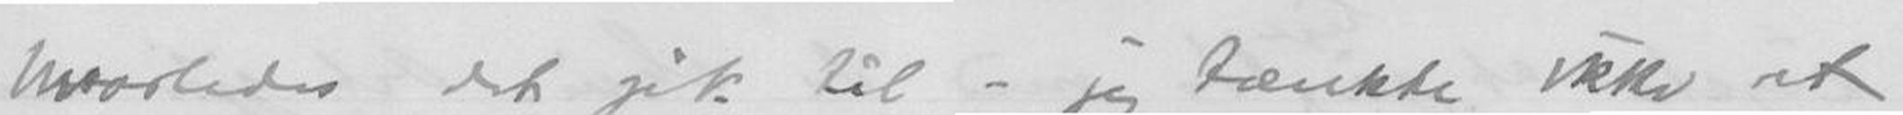hvorledes det gik til - jeg tænkte ikke et
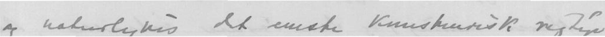og naturligvis det eneste kunstnerisk rigtige
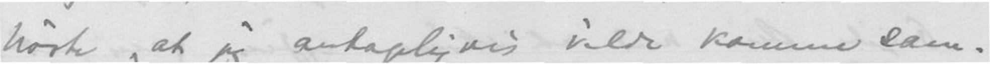hørte, at jeg antageligvis vilde komme sam-
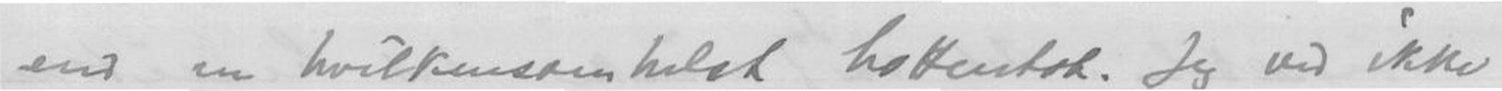end en hvilkensomhelst hottentot. Jeg ved ikke
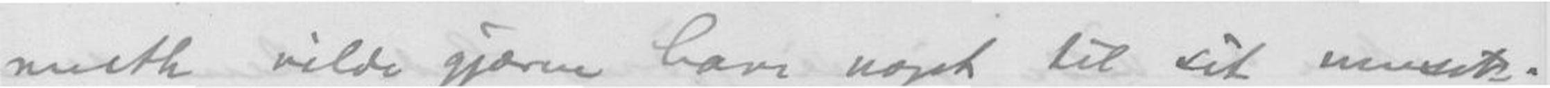muth vilde gjærne have noget til sit musik-
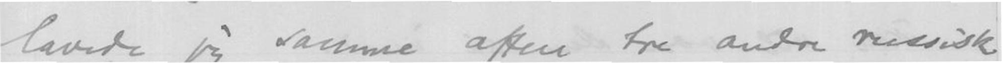lavede jeg samme aften tre andre russisk
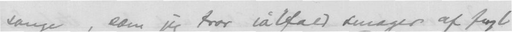sange, som jeg tror ialfald smager af fugl
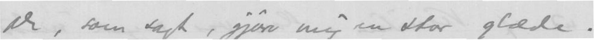De, som sagt, gjøre mig en stor glæde.
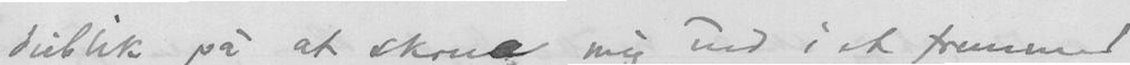Øieblik på at skru mig ind i et fremmed
